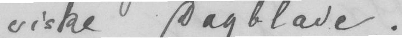viske Dagblade.
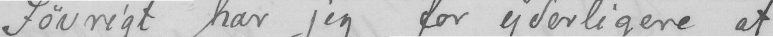Jøvrigt har jeg for yterligere at
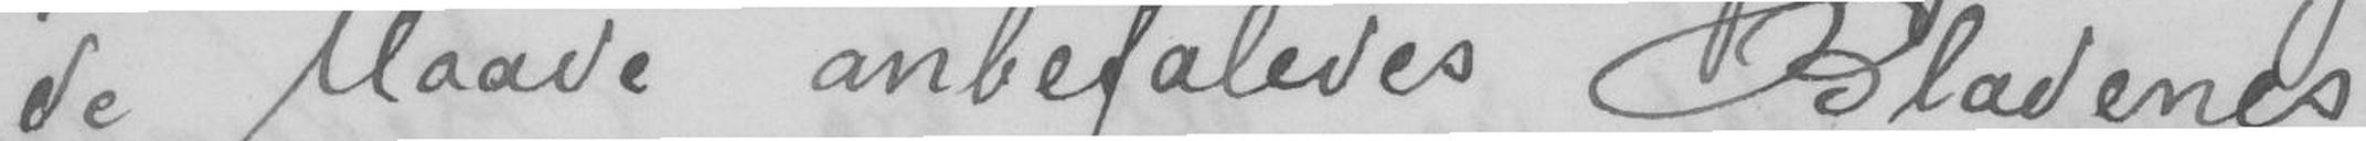de Maade anbefaledes Bladenes
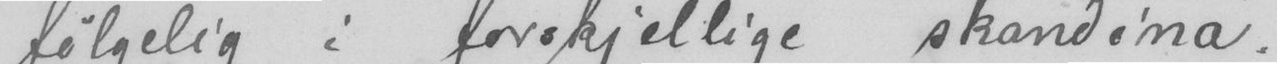følgelig i forskjellige skandina
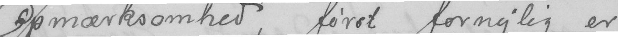Opmærksomhed, først fornylig er
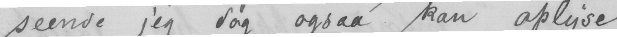seende jeg dog ogsaa kan oplyse
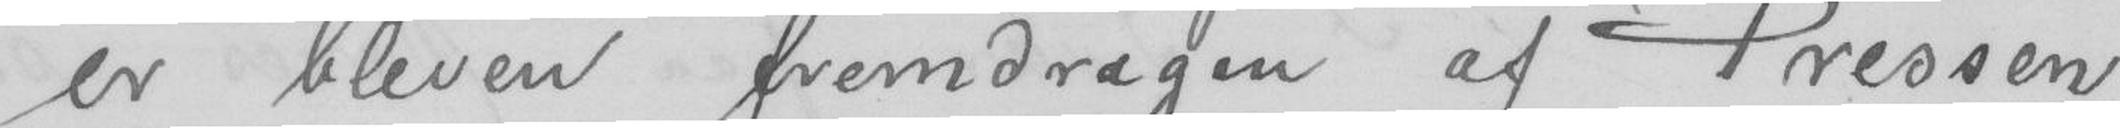er bleven fremdragen af Pressen
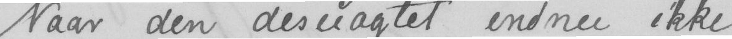Naar den desuagtet endnu ikke
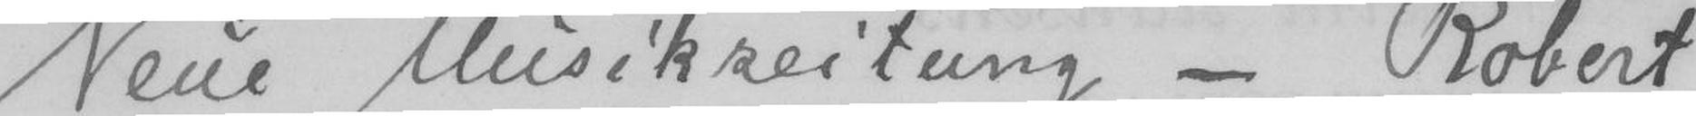Neue Musikreiting - Robert
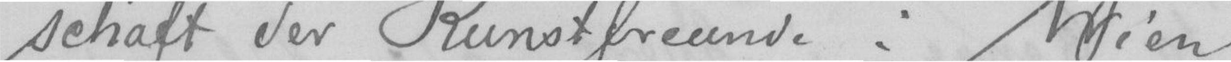schaft der Kunstfreunde i Wien
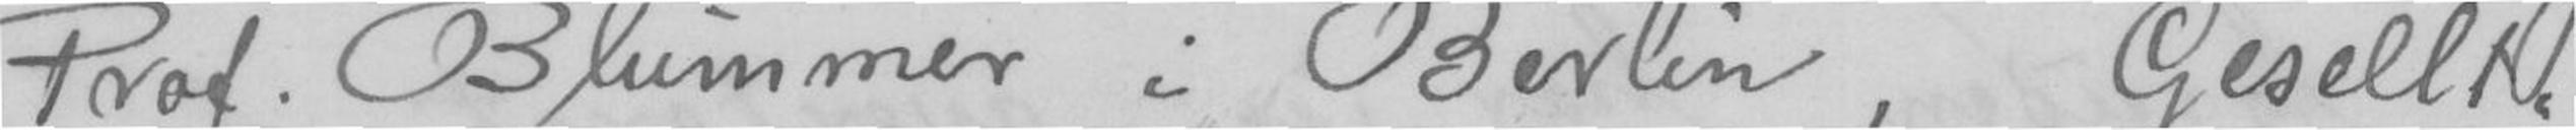Prof. Blummer i Berlin, Gesellt-
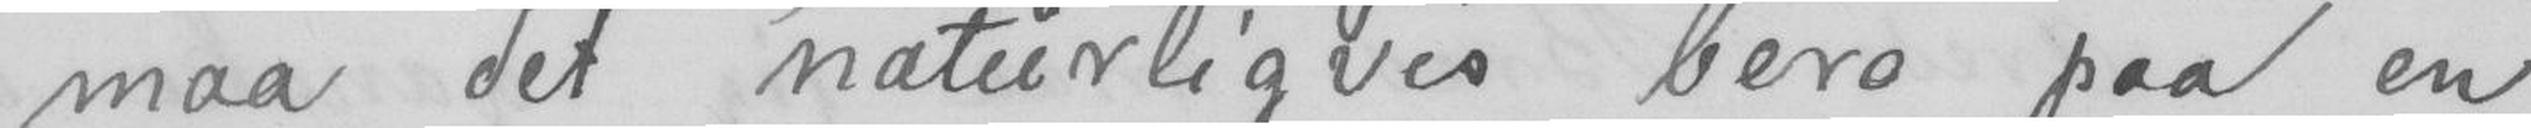maa det naturligvis bero paa en
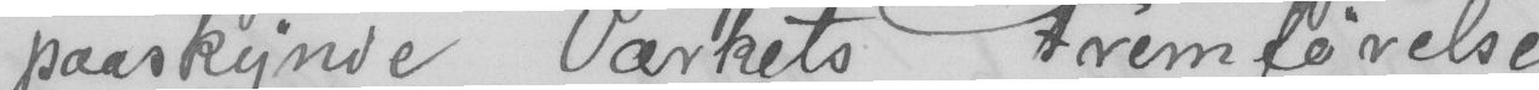paakynde Værkets Fremførelse
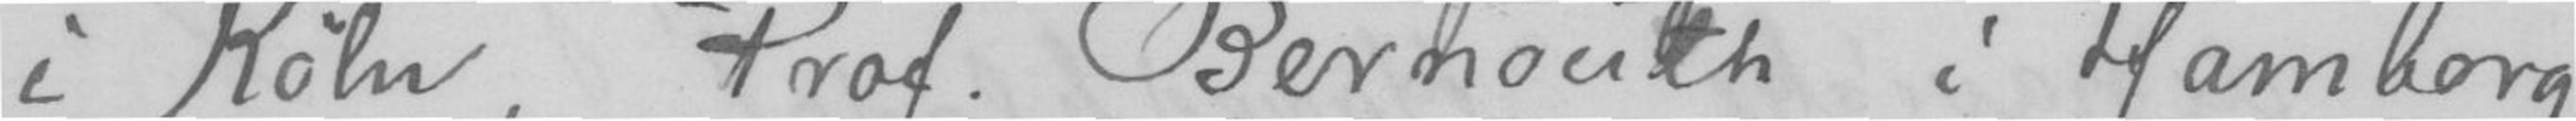i Køln, Prof. Bernouth i Hamborg
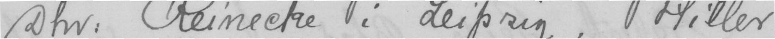Dher Reinecke i Leiprig, Hiller
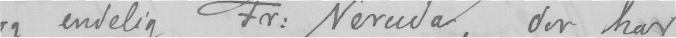og endelig Fr. Neruda, der har
